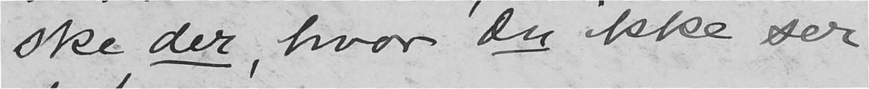ske der, hvor du ikke ser
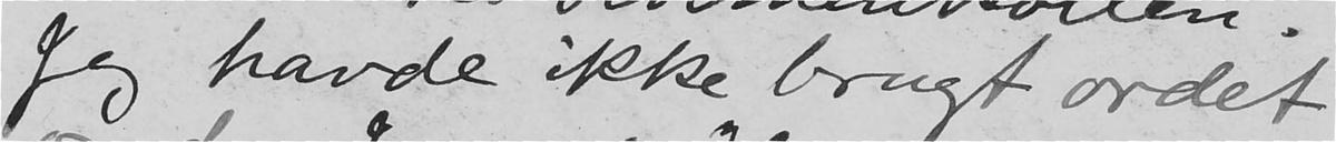Jeg havde ikke brugt ordet
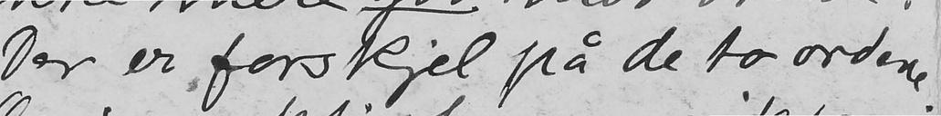Der er forskjel på de to ordene.
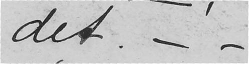det. - -
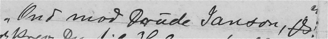"Ond mod Drude Janson, jeg"
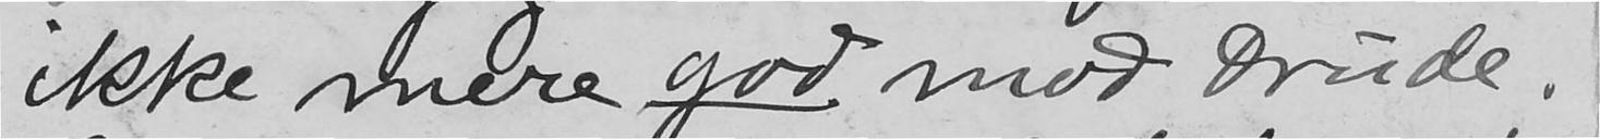ikke mere god mod Drude.
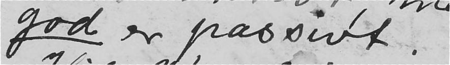god er passivt.
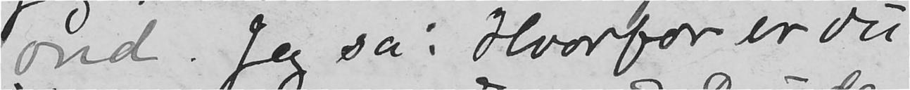ond. Jeg sa: Hvorfor er du
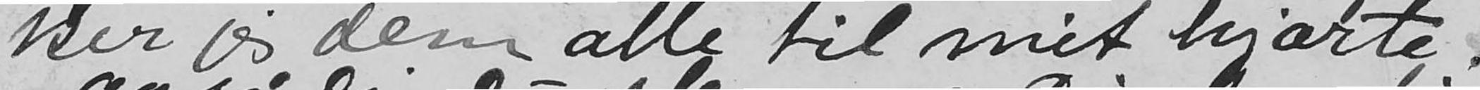ter jeg dem alle til mit hjærte.
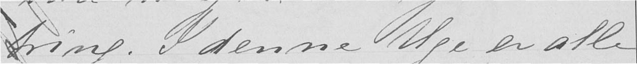tring. I denne Uge er alle
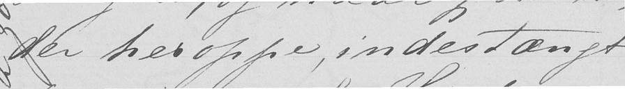der heroppe, indestængt
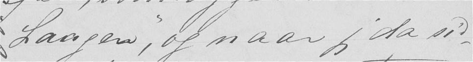Laugen, og naar jeg da sid-
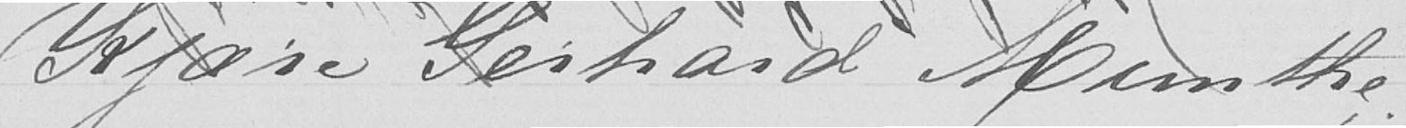Kjære Gerhard Munthe
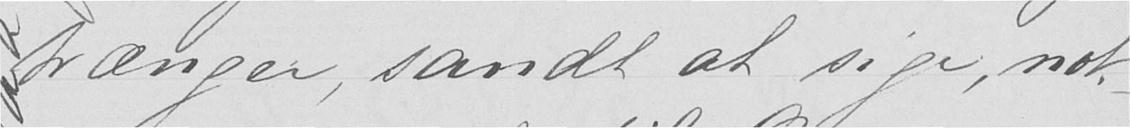Længer, sandt at sige, nok-
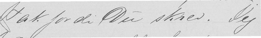Tak fordi Du skrev. Jeg
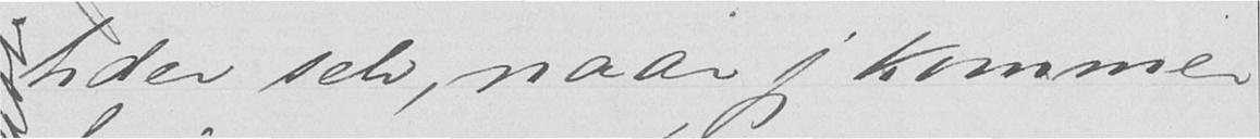tider selv, naar jeg kommer
